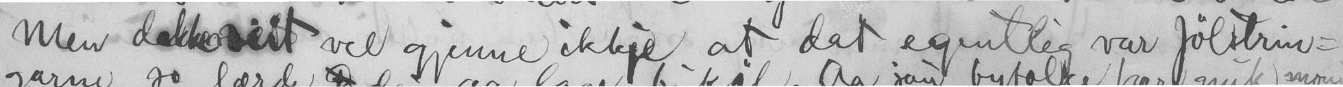Men dokke veit vel gjenne ikkje at dat egentlig var Jølstrin-
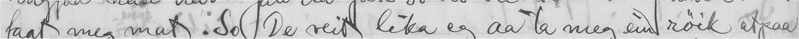faat me mat. So De veit lika eg aa ta meg ein røik atpaa
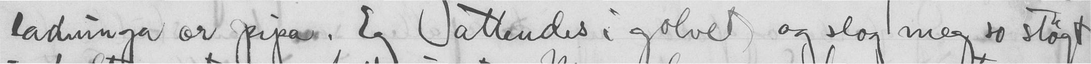ladninga or pipa. Eg attendes i golvet, og slog meg so støgt
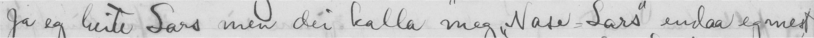Ja eg heite Lars med dei kalla meg "Nase-Lars" endaa eg mest
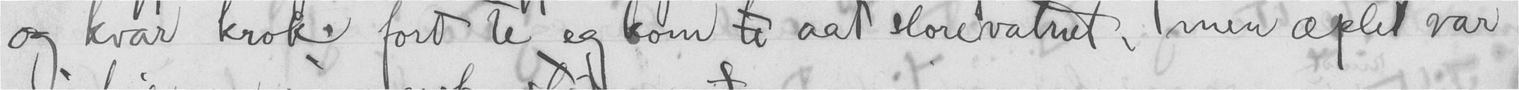og kvar krok fort te eg kom  aat slorevatnet, men æplet var
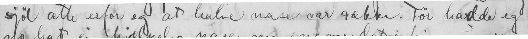sjøl atte erfor eg at halve nase var vække Før havde eg
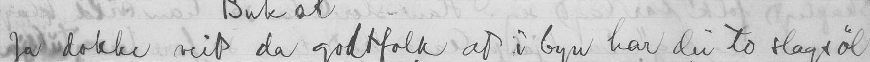Ja dokke veit da godtfolk at i byn har du to slags øl
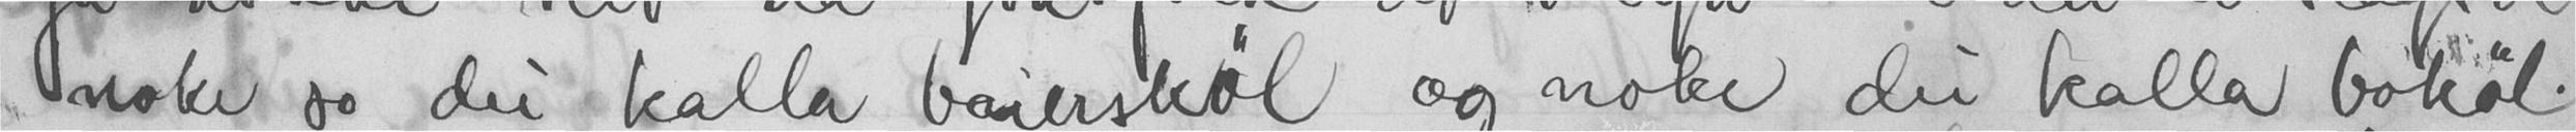noke so du kalle beierskøl og noke du kalle bokøl.
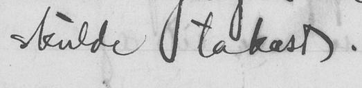skulde takast.
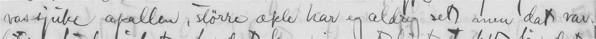vassjuke apallen større æple har eg aldreg set men dat var
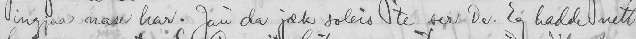ingjaa nase har. Jau da jæk soleis te ser De. Eg hadde nett
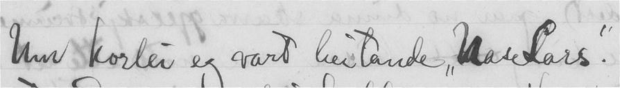Um korlei eg vart heitande "NaseLars".
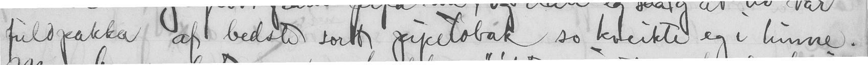fuldpakke af bedste sort pipetobak so kveikte og i hinne.
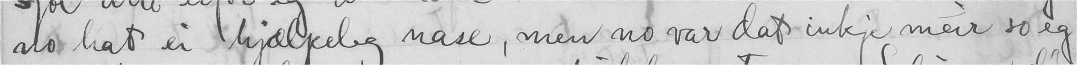no hat ei hjælpeleg nase, men no var dat inkje mere so eg
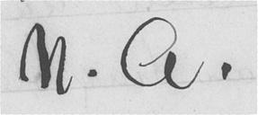N. A.
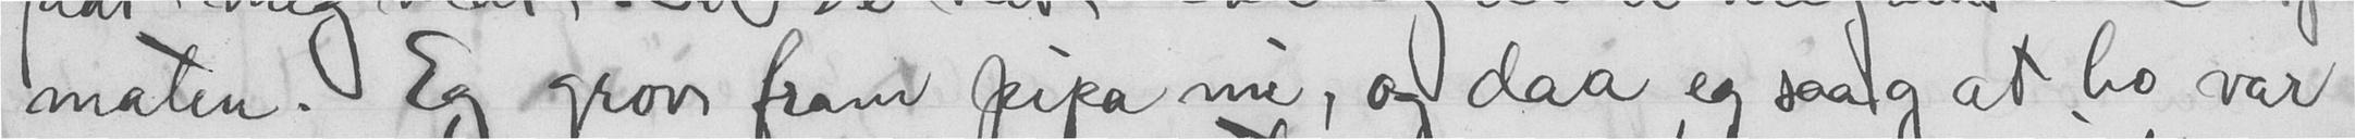maten. Eg grov fram pipa mi, og daa eg saag at ho var
